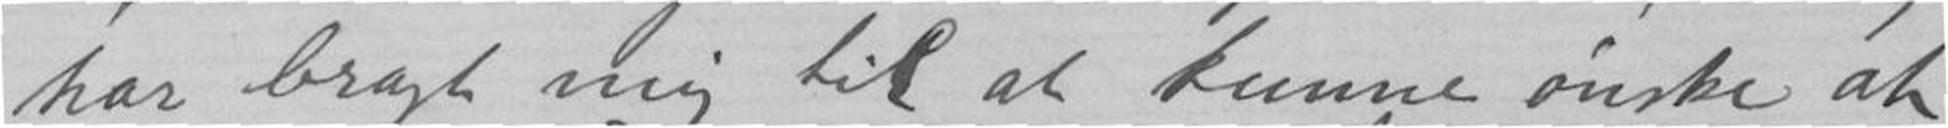har bragt mig til at kunne ønske at
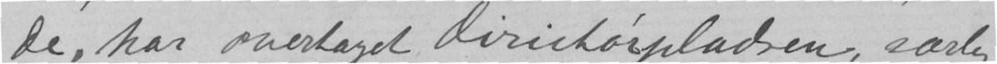De, har overtaget Dirictørpladsen, særlig
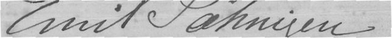Emil Jähnigen
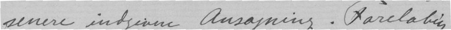senere indgivne Ansogning. Foreløbig
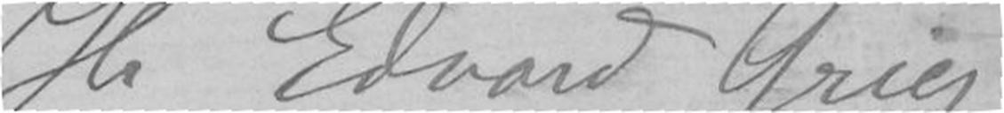Hr Edvard Grieg
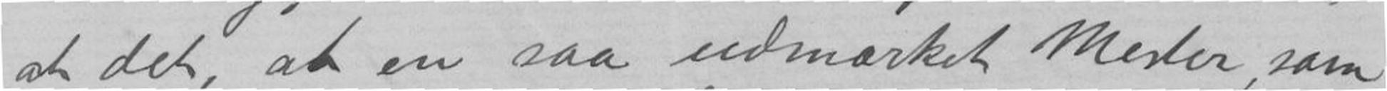at det, at en saa udmærket Mester, som
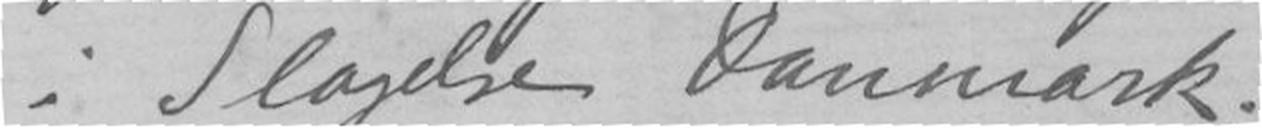i Slagelse Danmark.
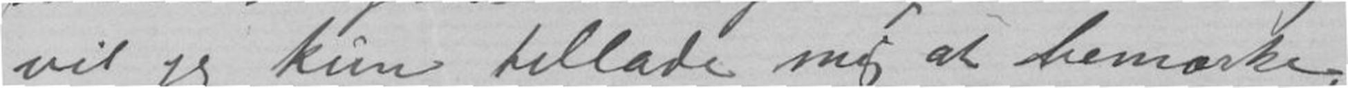vil jeg kun tillade mig at bemærke,
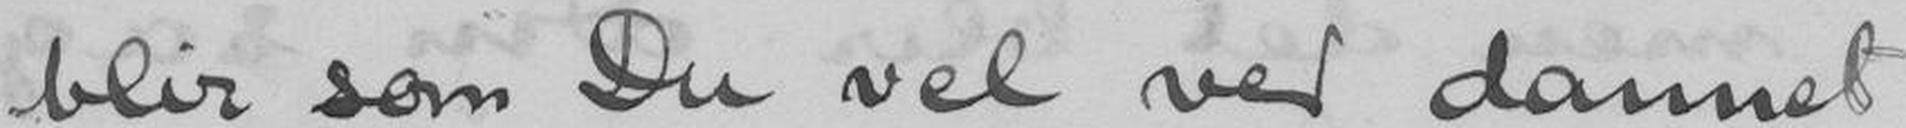blir som Du vel ved dannet
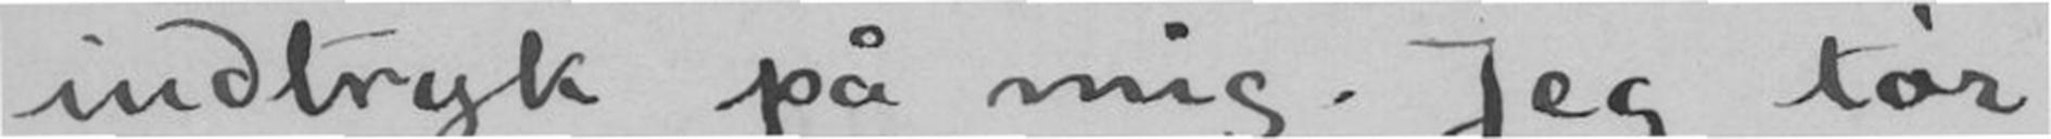indtryk på mig. Jeg tør
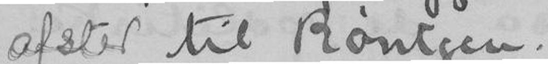afsted til Röntgen.
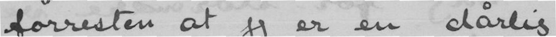forresten at jeg er en dårlig
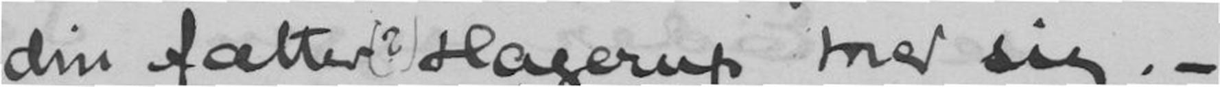din fætter(?) Hagerup med sig. -
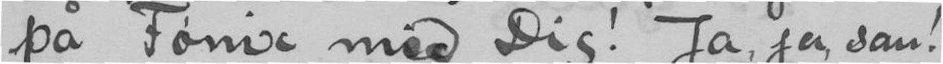på Fønix med Dig! Ja, ja, san!
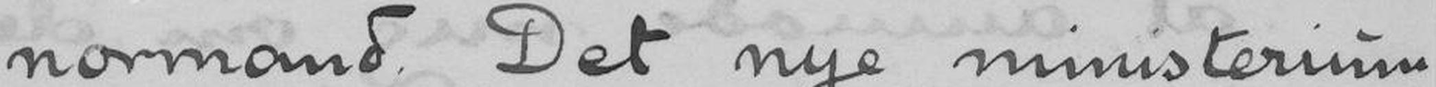nordmand. Det nye ministerium
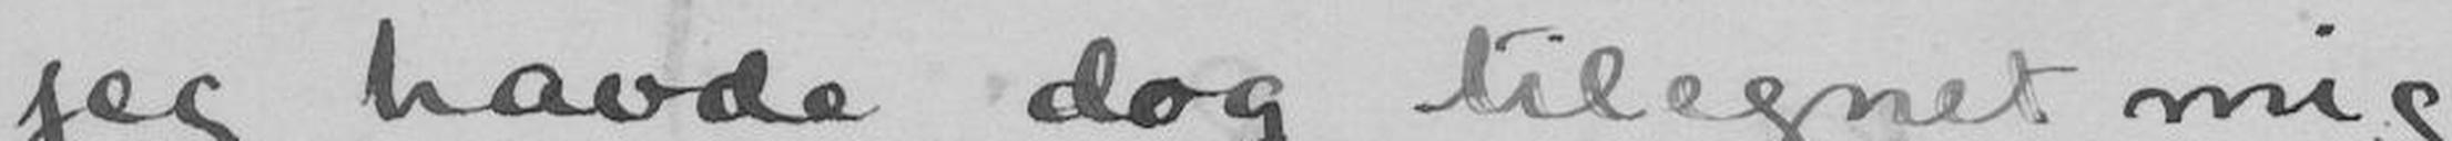jeg havde dog tilegnet mig
